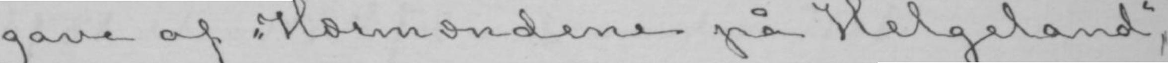gave af «Hærmændene på Helgeland»,
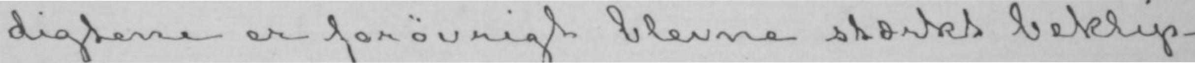digtene er forøvrigt blevne stærkt beklip-
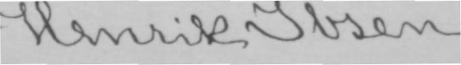Henrik Ibsen.
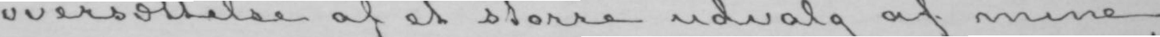oversættelse af et større udvalg af mine
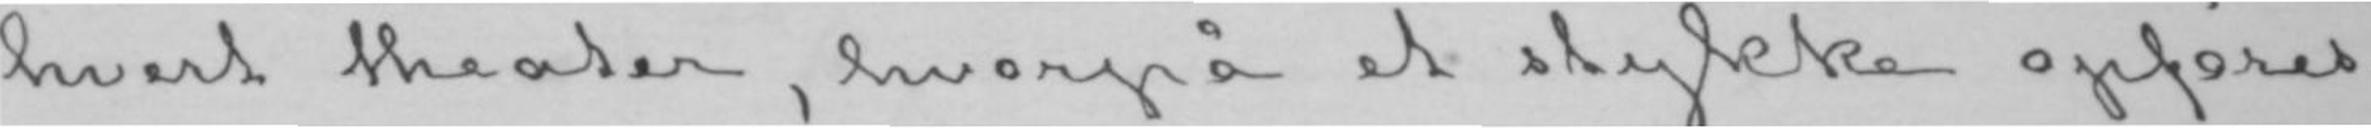hvert theater, hvorpå et stykke opføres,
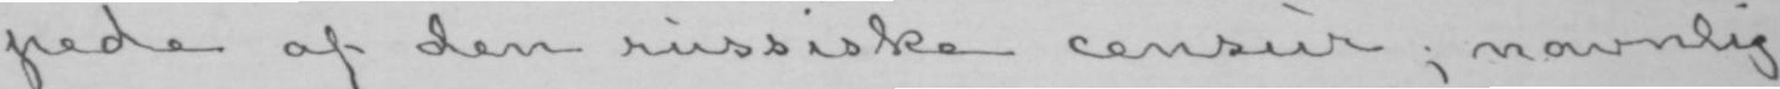pede af den russiske censur; navnlig
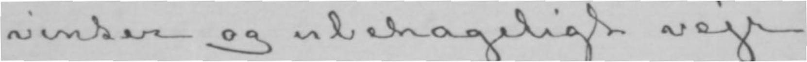vinter og ubehageligt vejr.
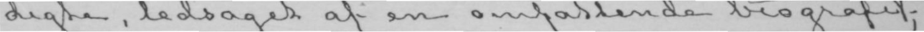digte, ledsaget af en omfattende biografi
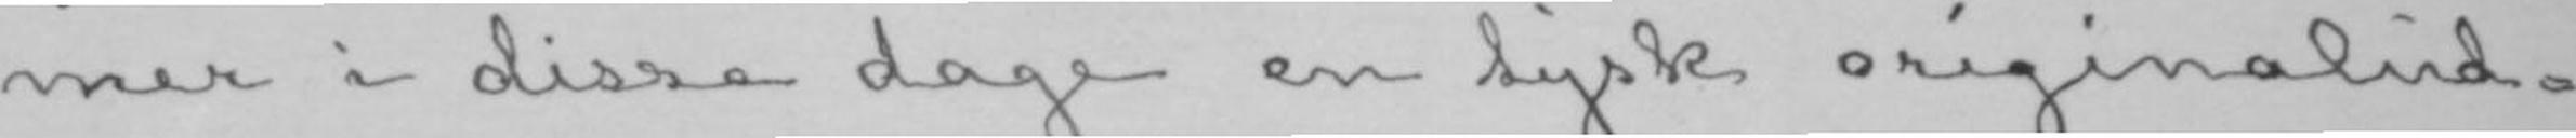mer i disse dage en tysk originalud-
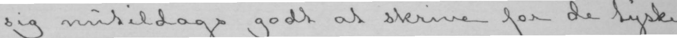sig nutildags godt at skrive for de tyske
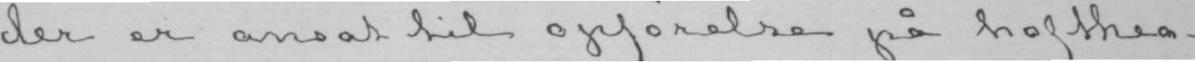der er ansat til opførelse på hofthea-
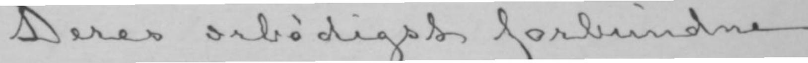Deres ærbødigst forbundne
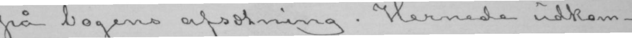på bogens afsætning. Hernede udkom-
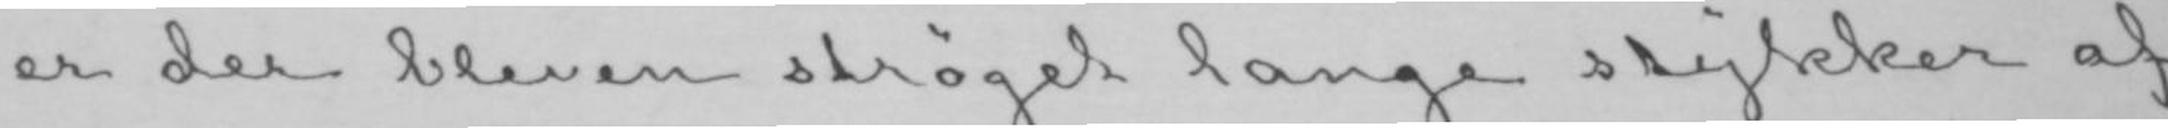er der bleven strøget lange stykker af
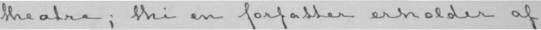theatre; thi en forfatter erholder af
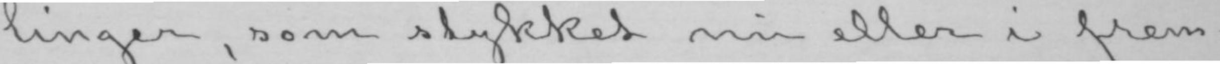linger, som stykket nu eller i frem-
